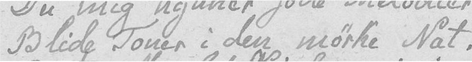Blide Toner i den mørke Nat.
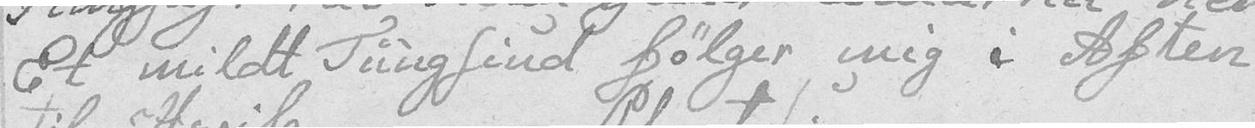Et mildt Tungsind følger mig i Aften
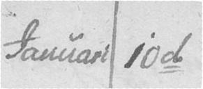Januari 10de
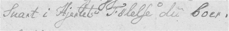Snart i Hjertets Følelse du boer.
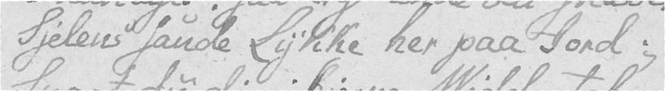Sjelens sande Lykke her paa Jord.
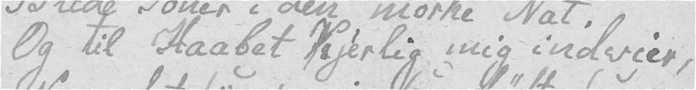Og til Haabet Kjerlig mig indvier,
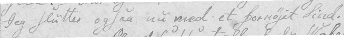Jeg slutter ogsaa nu med et fornøjet Sind.
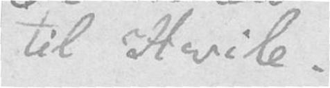til Hvile.
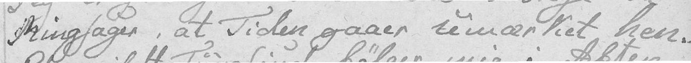Ringsager, at Tiden gaaer umærket hen.
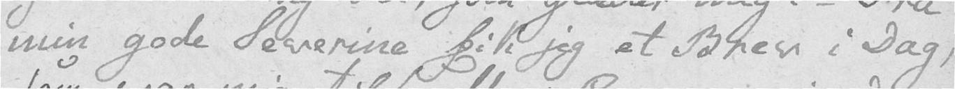min gode Severine fik jeg et Brev i Dag,
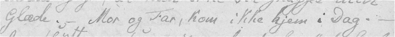Glæde. – Mor og Far, kom ikke hjem i Dag. –
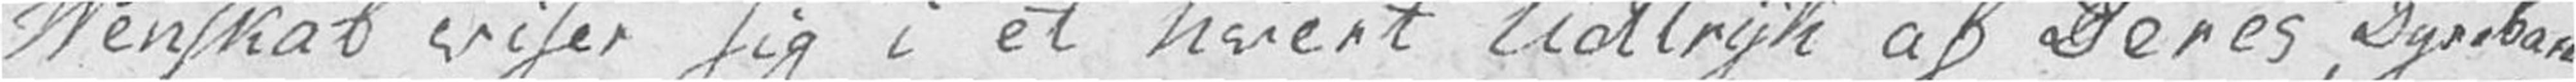Venskab viser sig i et hvert Udtryk af Deres Dyrebare
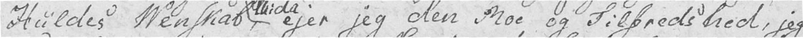Huldes Venskab, thi da ejer jeg den Roe og Tilfredshed, jeg
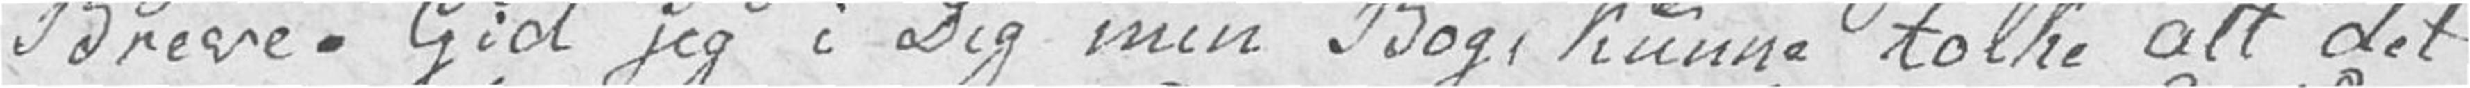Breve. Gid jeg i Dig min Bog, kunne tolke alt det
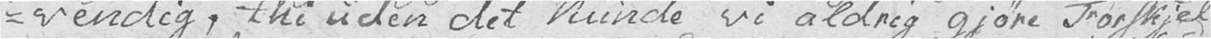-vendig, thi uden det kunde vi aldrig gjøre Forskjel
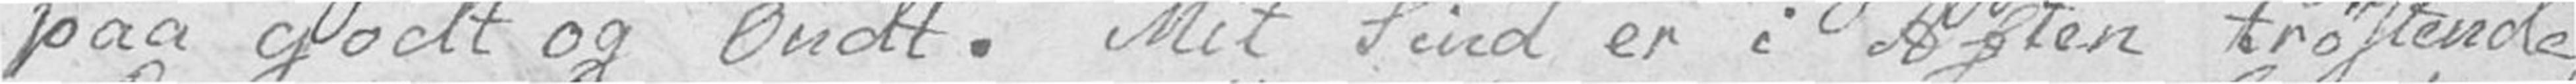paa godt og Ondt. Mit Sind er i Aften trøstende,
